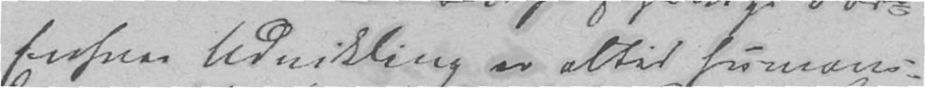Enhver Udvikling er altid humani-
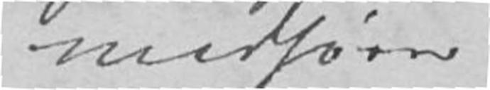medfører
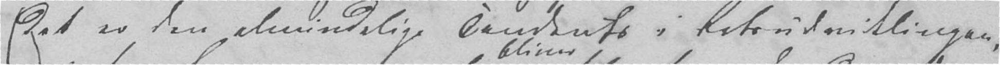det er den almindelige Tendents i Retsudviklingen,
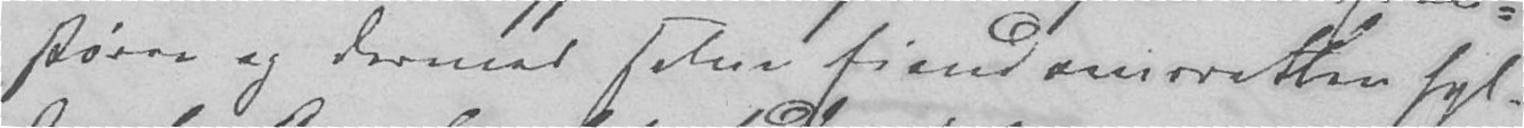større og dermed selve Eiendomsretten fyl-
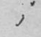i
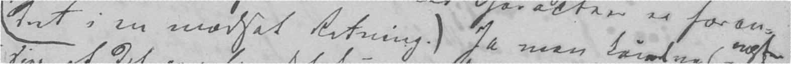dret i en modsat Retning.) Ja man kunde vel næsten
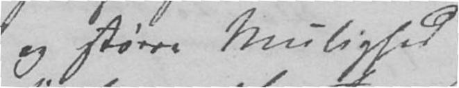og større Mulighed
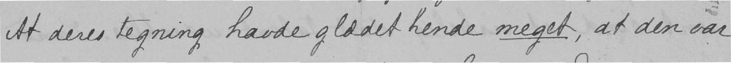At deres tegning havde glædet hende meget, at den var
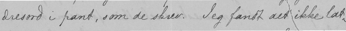æresord i pant, som de skrev. Jeg fandt det ikke lat-
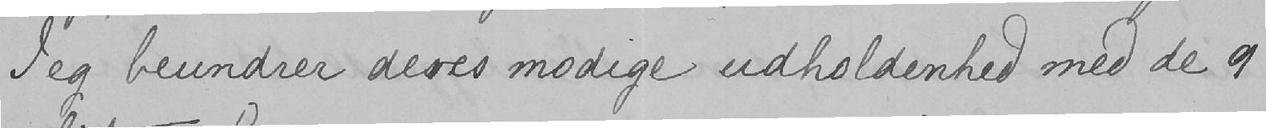Jeg beundrer deres modige udholdenhed med de 9
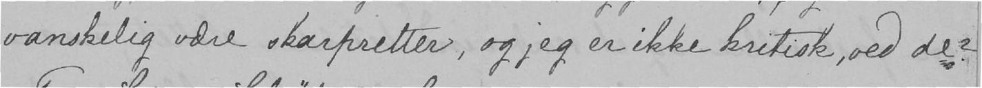vanskelig være skarpretter, og jeg er ikke kritisk, ved de?
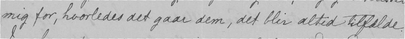mig for, hvorledes det gaar dem, det blir altid tilfælde.
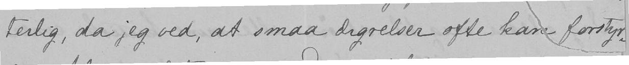terlig, da jeg ved, at smaa ærgrelser ofte kan forstyr-
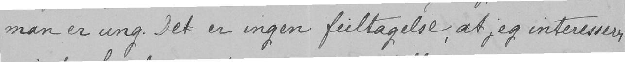man er ung. Det er ingen feiltagelse, at jeg interesserer
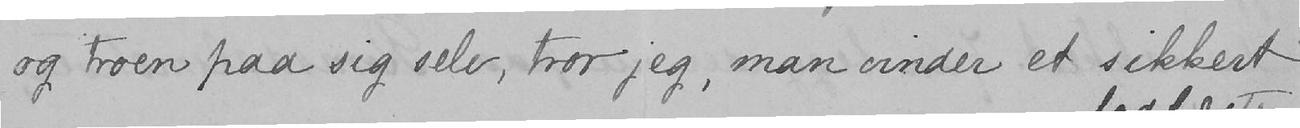og troen paa sig selv, tror jeg, man vinder et sikkert
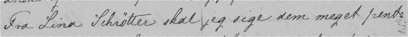Fra Lina Schrøtter skal jeg sige dem meget pent:
